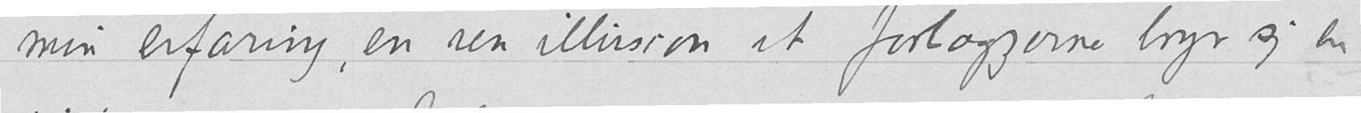min erfaring, en ren illusion at forlæggerne bryr sig en
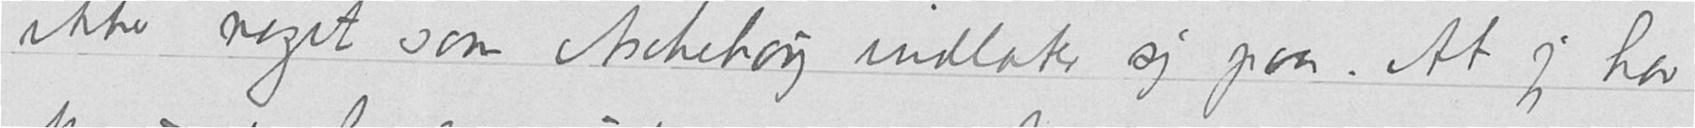ikke noget som Aschehoug indlater sig paa. At jeg har
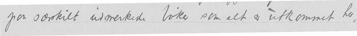paa særskilt udmerkede bøker som alt er utkommet her,
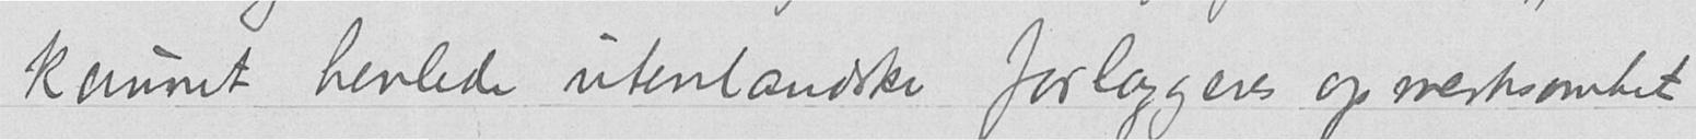kunnet henlede utenlandske forlæggeres opmerksomhet
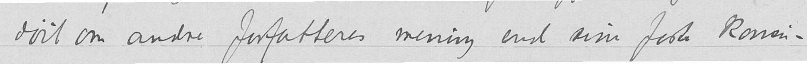døit om andre forfatteres mening end sine faste konsu-
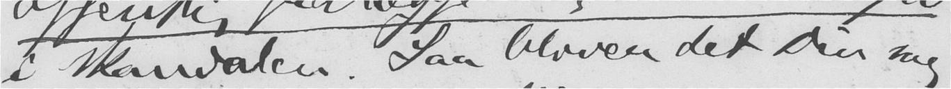i skandalen. Saa bliver det Din sag
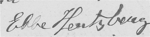Ebbe Hertzberg
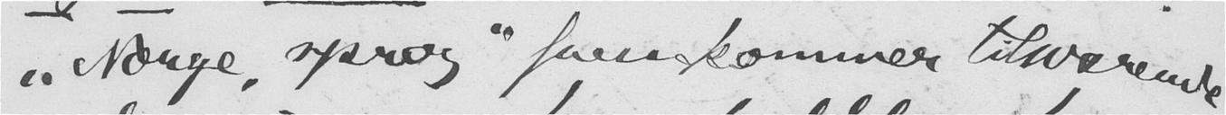"Norge, sprog" fremkommer tilsvarende
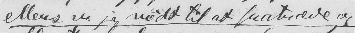ellers er jeg nødt til at fratræde og
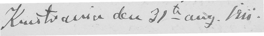Kristiania den 31te aug. 1911.
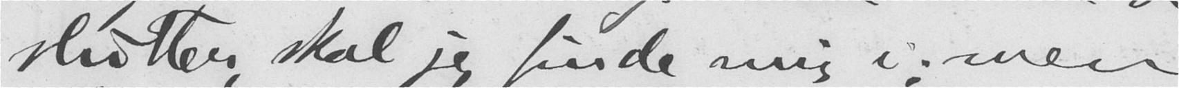slutter, skal jeg finde mig i; men
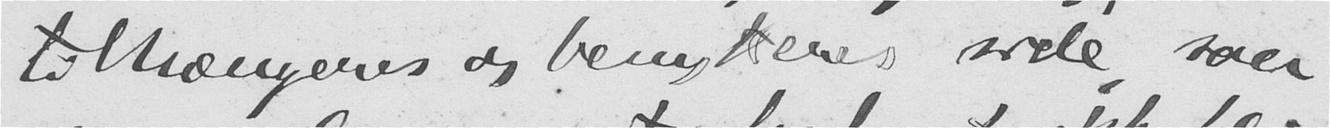tilhængeres og benytteres side, saa
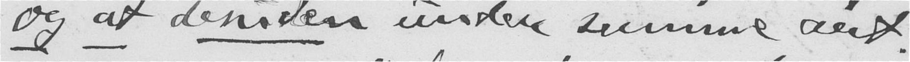og at desuden under summe art.
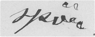spør.
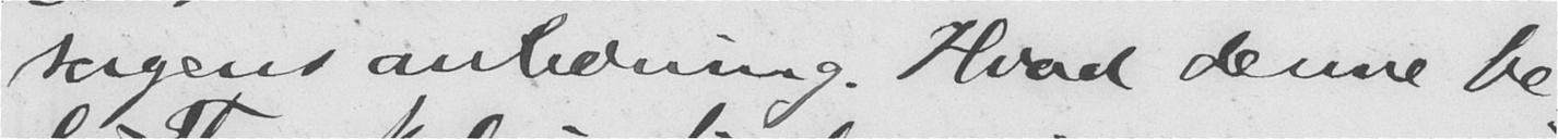sagens anledning. Hvad denne be-
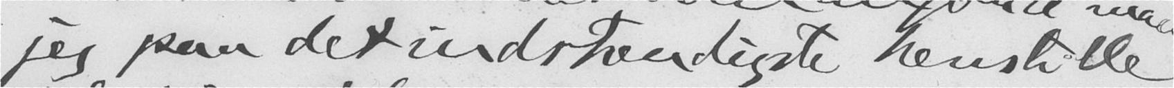jeg paa det indstændigste henstille
